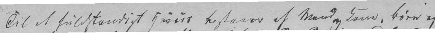Til et fuldstændigt Huus bestaaer af Mand og Kone, Børn og
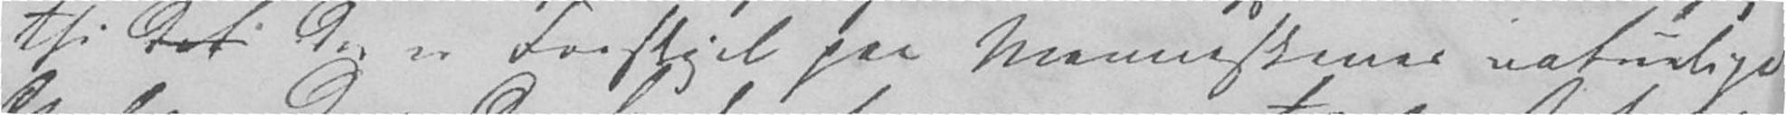thi det der er Forskjel paa Menneskenes naturlige
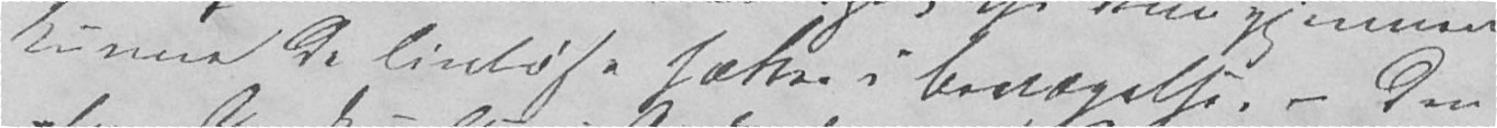kunne de livløse sættes i Bevægelse. - Den
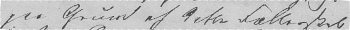paa Grund af dette Fællesskab
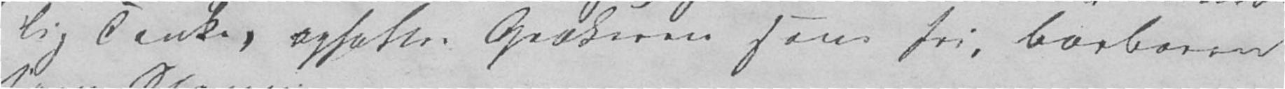lig Tanke, opfatter Grækeren som fri, Barbarerne
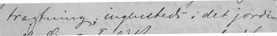tragtning; ingenstede i det jordi-
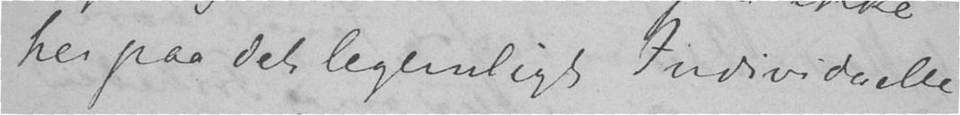her paa det legemligt Individuelle
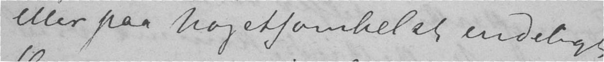eller paa Nogetsomhelst endeligt
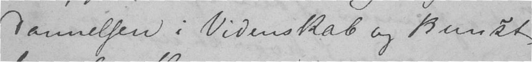dannelsen i Videnskab og Kunst
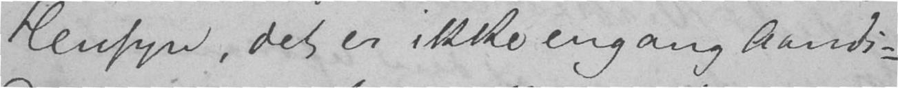Hensyn, det er ikke engang Aands-
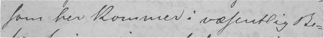som her kommer i væsentlig Be-
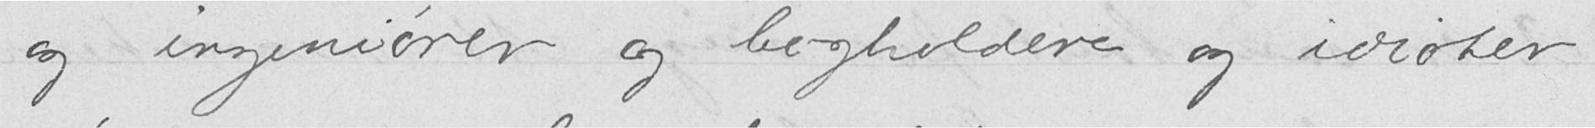og ingeniører og bogholdere og idioter
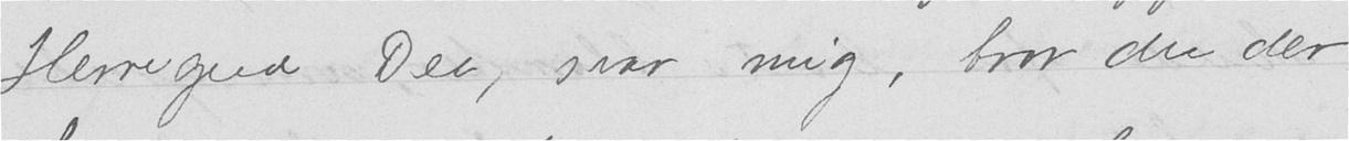Herregud Dea, svar mig, tror du der
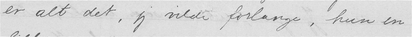er alt det, jeg vilde forlange, kun en
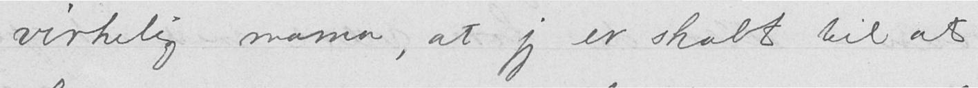virkelig mama, at jeg er skabt til at
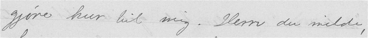gjøre kur til mig. Herre du milde,
